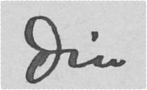Din
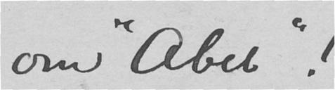om "Abel"!
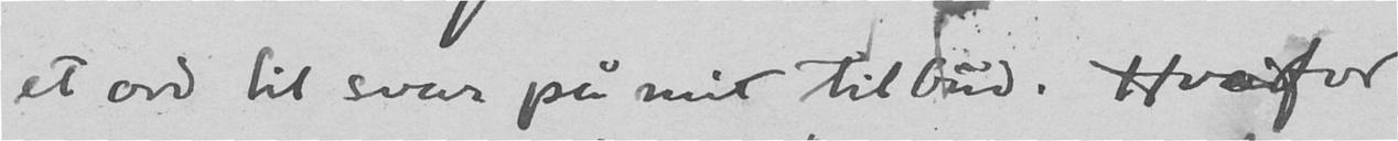et ord til svar på mit tilbud. Hvorfor
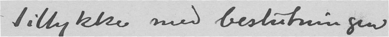Tillykke med beslutningen
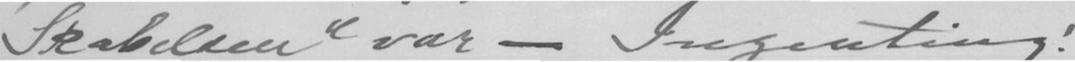"Skabelsen" var - Ingenting!
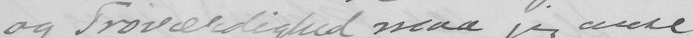og Troværdighed maa jeg anse
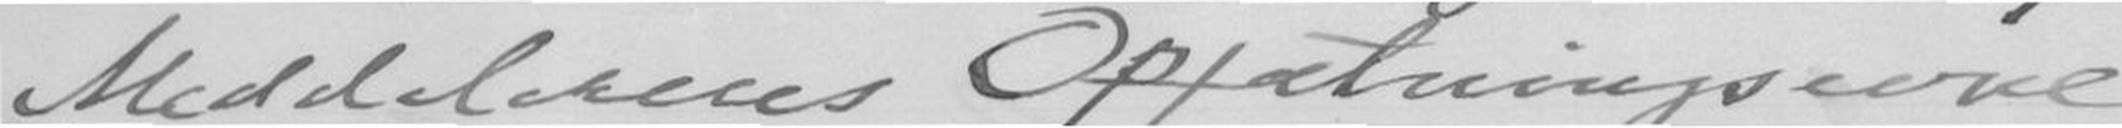Meddelernes Opfatningsevne
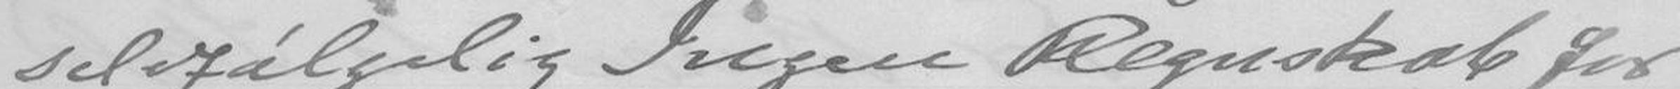selvfølgelig Ingen Regnskab for
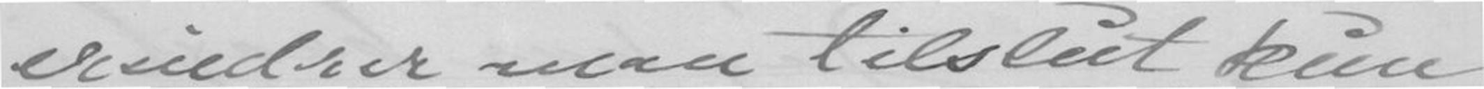erindrer man tilslut kun
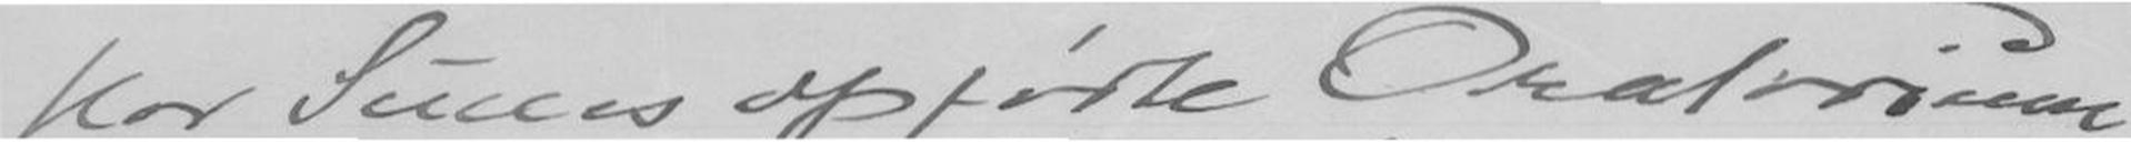stor Succes opførte Oraturium
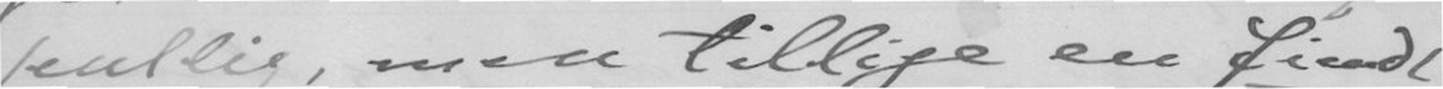fentlig, men tillige en fiendt-
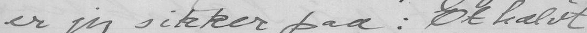er jeg sikker paa: Et halvt
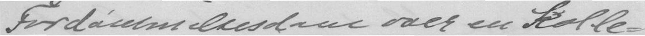Fordømmelsesdom over en Kolle-
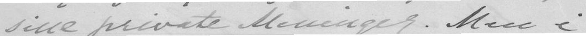sine private Meninger. Men i
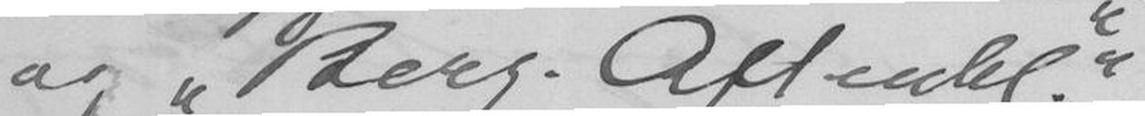af "Berg. Aftenbl."
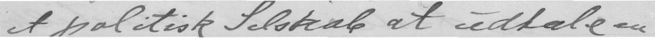et politisk Selskab at udtale en
